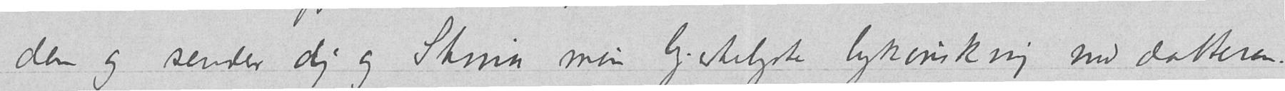den og sender dig og Stina min hjerteligste lykønskning med datteren.
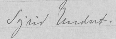Sigrid Undset.
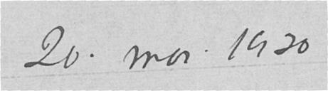20. mai. 1930
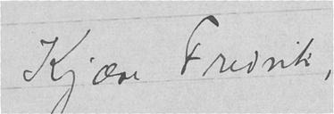Kjære Fredrik,
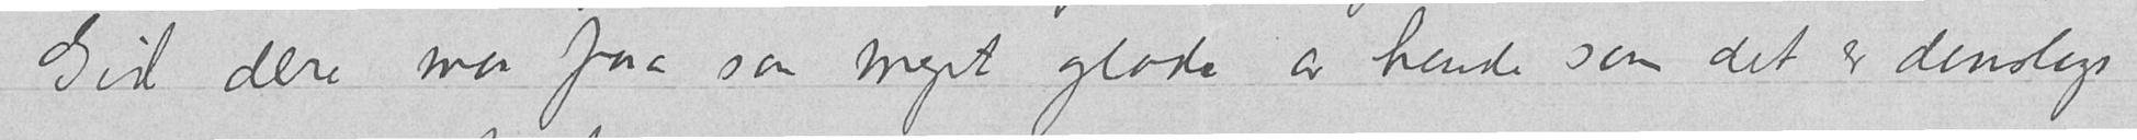Gid dere maa faa saa meget glæde av hende som det er denslags
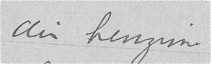din hengivne
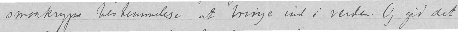smaakryps bestemmelse at bringe ind i verden. Og gid det
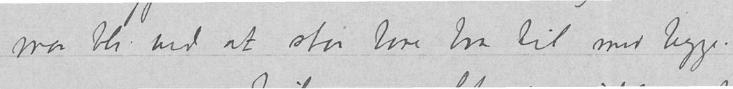maa bli ved at staa bare bra til med begge.
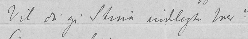Vil du gi Stina indlagte brev?
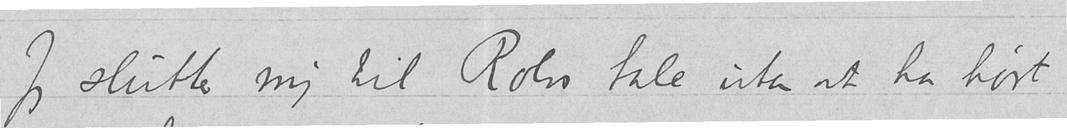Jeg slutter mig til Rolvs tale uten at ha hørt
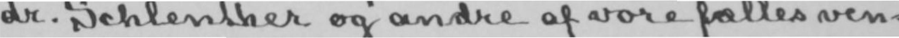dr. Schlenther og andre af vore fælles ven-
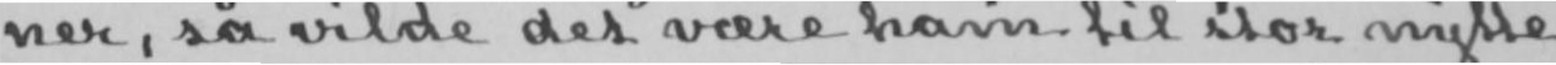ner, så vilde det være ham til stor nytte
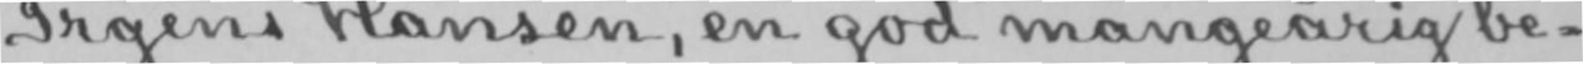Irgens Hansen, en god mangeårig be-
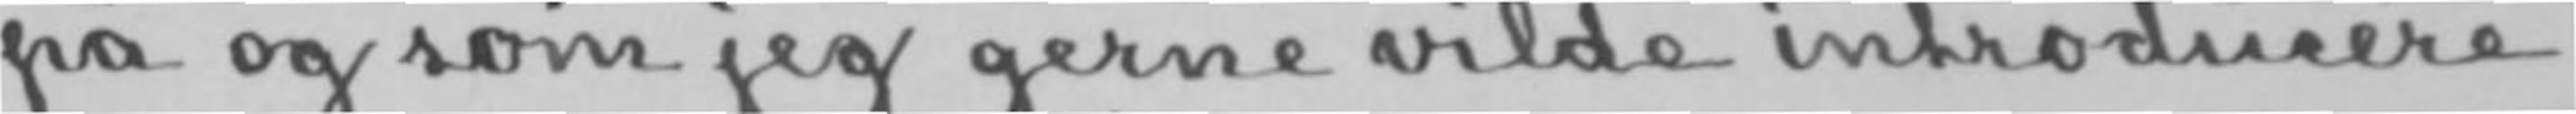på og som jeg gerne vilde introducere
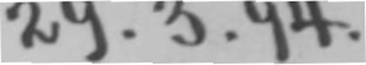29.3.94.
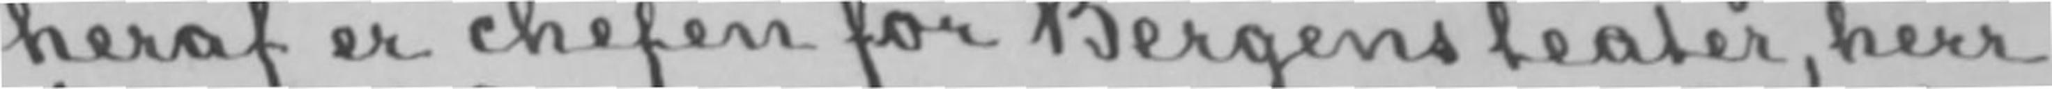heraf er chefen for Bergens teater, herr
